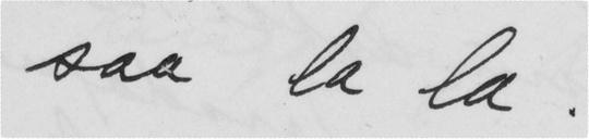saa la la.
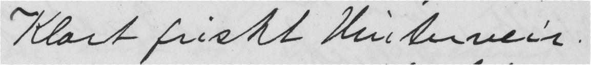Klart friskt Vinterveir.
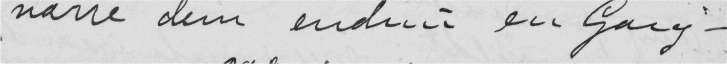narre dem endnu en Garg —
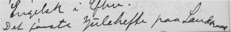Det første Julehefte paa Landsmaal
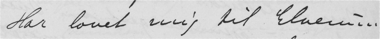Har lovet mig til Elverum
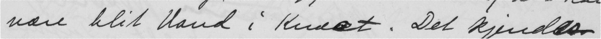være blit Vand i Knæet. Det kjendes
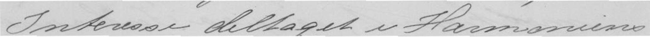Intresse deltaget i Harmoniens
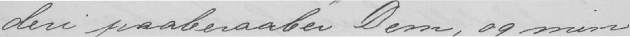den paaberaaber Dem, og min
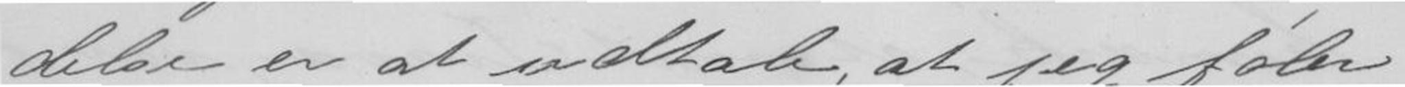delse er at udtale, at jeg føler
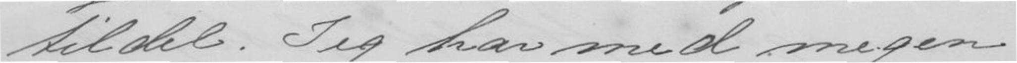tildels. Jeg har med megen
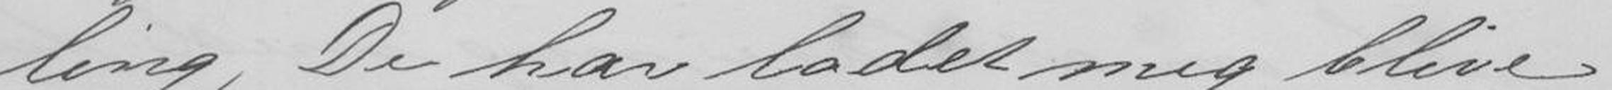ling, de har ladet mig blive
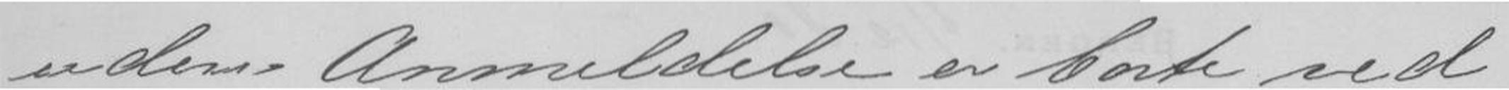uden Anmeldelse er borte ved
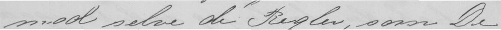med selve de Regler, som De
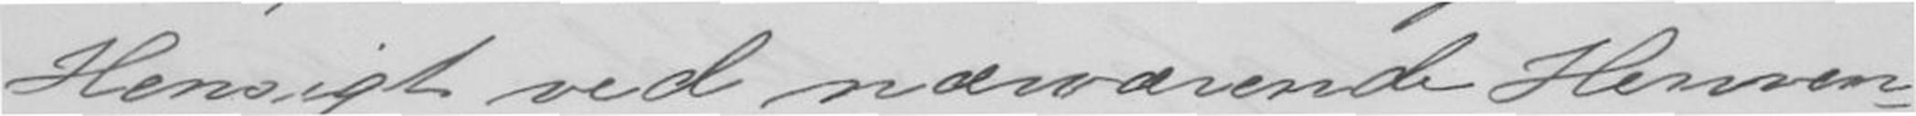Hensigt ved nærværende Henven-
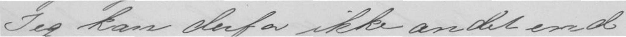Jeg kan derfor ikke andet
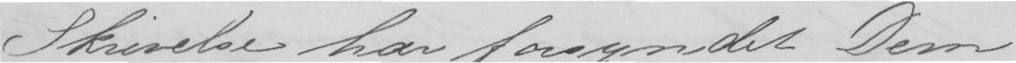Skrivelse har forsyndet Dem
